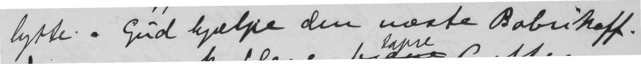lytte. Gud hjælpe den næste Bobrikoff.
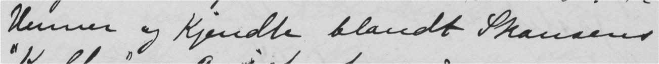Venner og Kjendte blandt Skansens
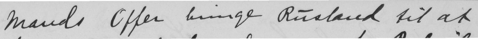Mands Offer bringe Rusland til at
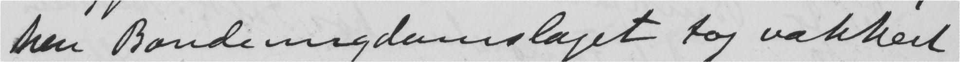Men Bondeungdomslaget tog vakkert
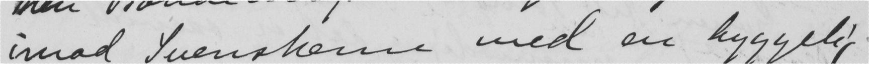imod Svenskerne med en hyggelig
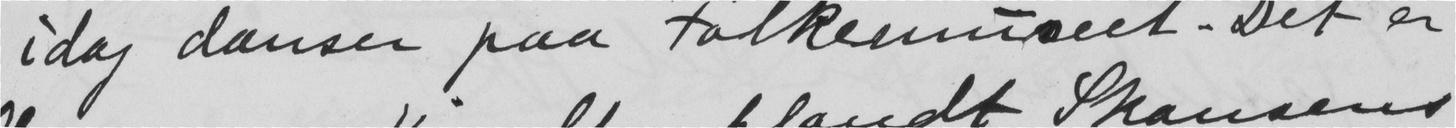idag danser paa Folkemuseet. Det er
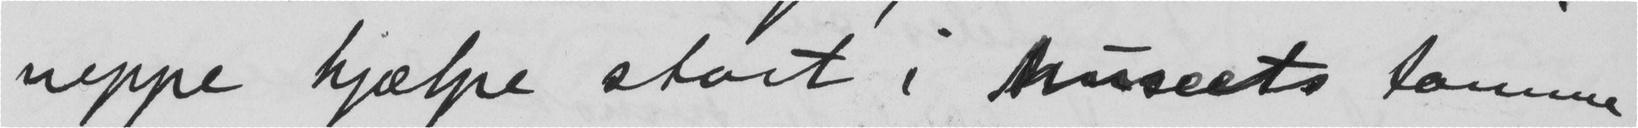neppe hjælpe stort i Museets tomme
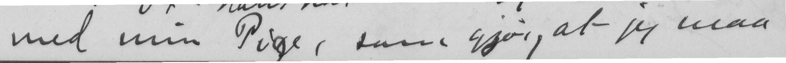med min Pige, som gjør, at jeg maa
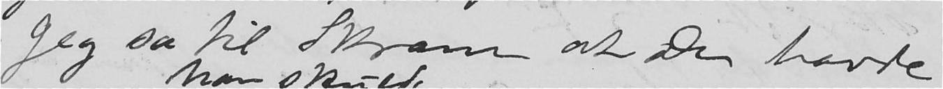Jeg ss til Skram at Du havde
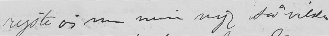rejste jeg nu min vej, så vilde
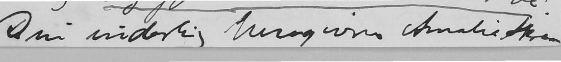Din inderlig hengivne Amalie Skram.
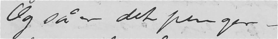Og så er det penger -
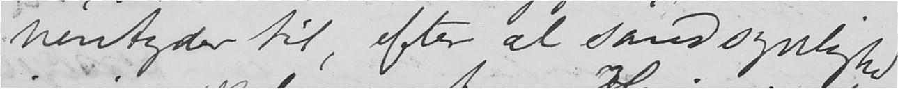hentyder til, efter al sandsynlighed
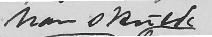han skulde
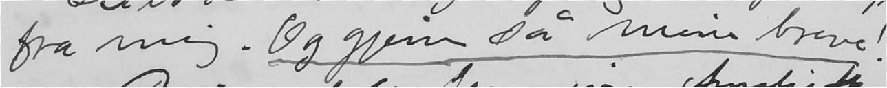fra mig. Og gjem så mine breve!
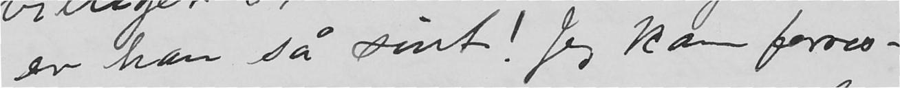er han så sint! Jeg kan forres-
